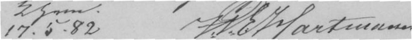17.5.82J. P. E. Hartmann
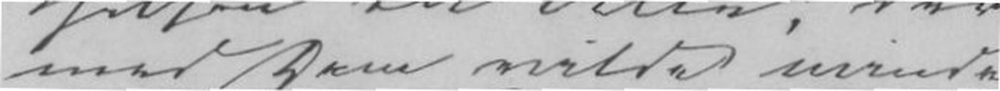med Dem vilde mindes
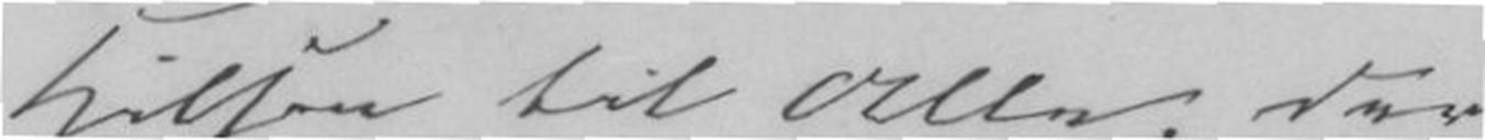Hilsen til Alle, der
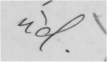ud.
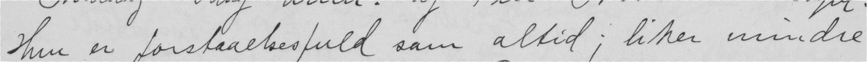Hun er forstaaelesfuld som altid; liker mindre
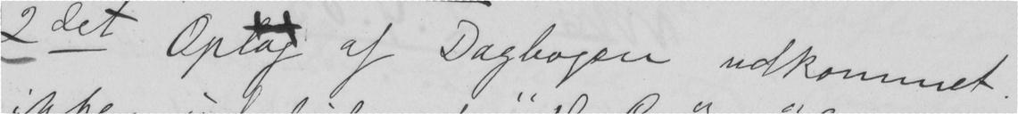2det Oplag af Dagbogen udkommet.
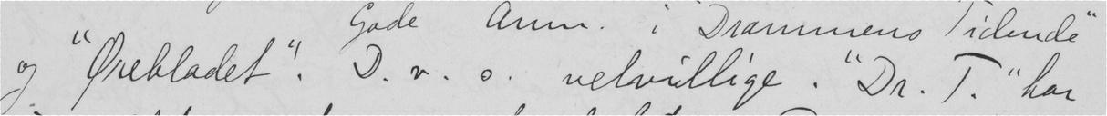og "Ørebladet". D. v. s. velvillige. "Dr. T." har
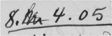8. 4.05
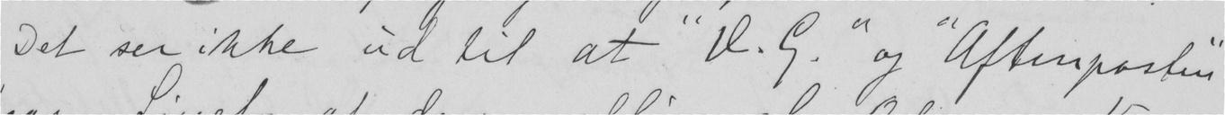Det ser ikke ud til at "V. G." og "Aftenposten"
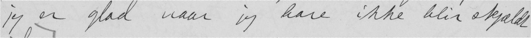Jeg er glad naar jeg bare ikke blir skjældt
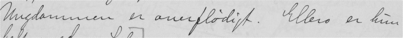Ungdommen er overflødigt. Ellers er hun
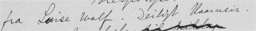fra Luise Wolf. Deiligt Vaarveir.
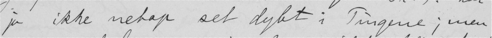jo ikke netop set dybt i Tingene; men
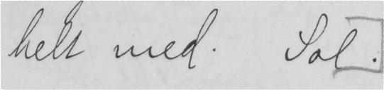helt med. Sol.
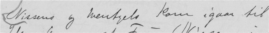Nissens og Wentzels kom igaar til
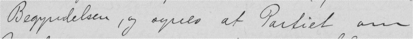Begyndelsen, og synes at Partiet om
